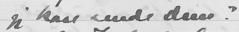jeg kan sende Dem?
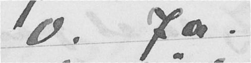O. Ja.
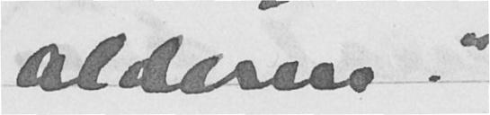alderen."
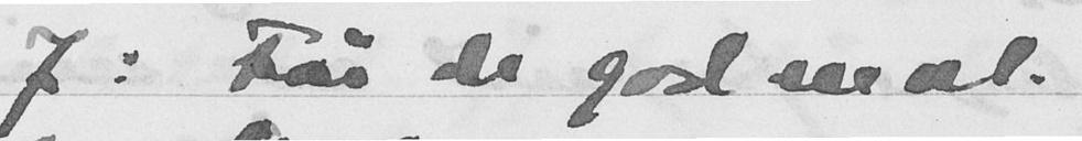J. Får de god mat.
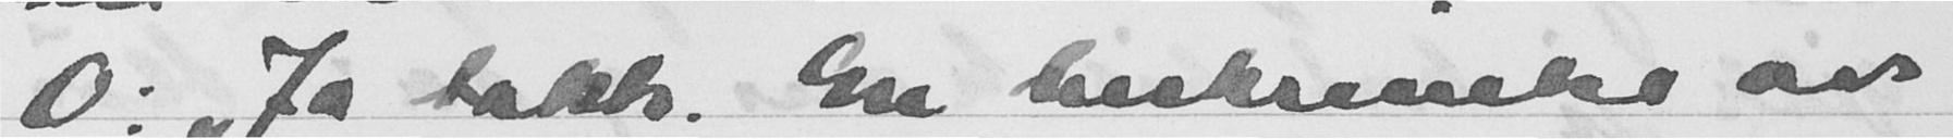O: "Ja takk. En beskrivelse av
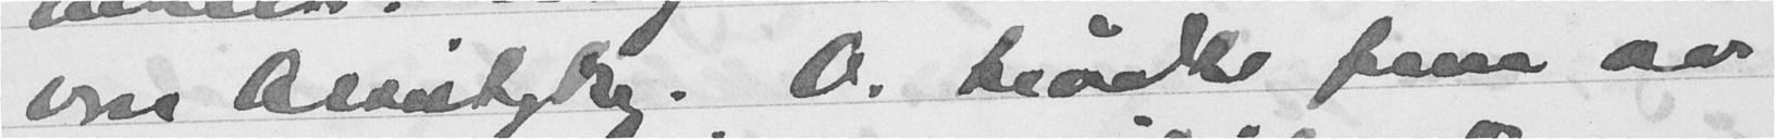von Ossietzky. O. trådte frem av
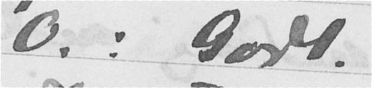O.: Godt.
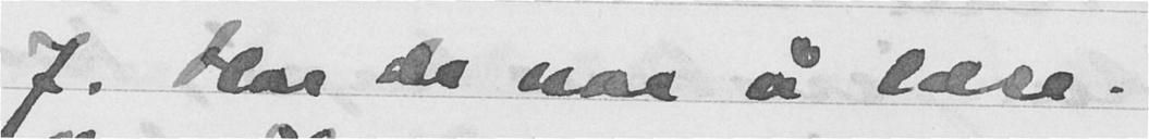J. Har de noe å læse.
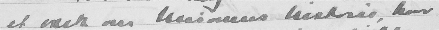et værk om anns historie, hvor
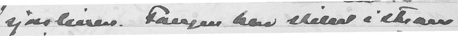sjøsliessen. Fangen har stillet i stram
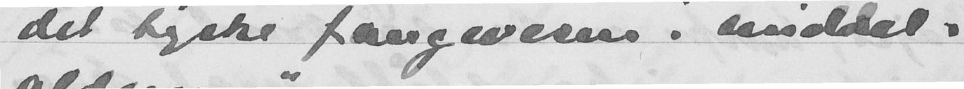det tyske fangevesen i middel-
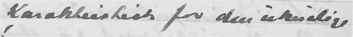Karakteristisk for den ukuelige
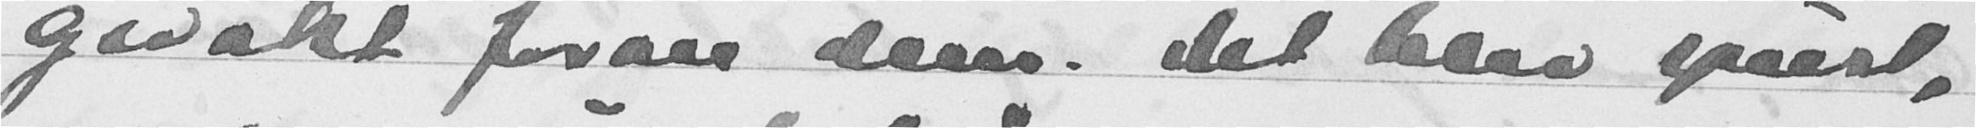givakt foran dem. Det blev spurt,
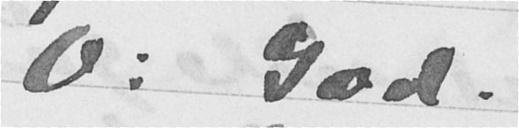O: God.
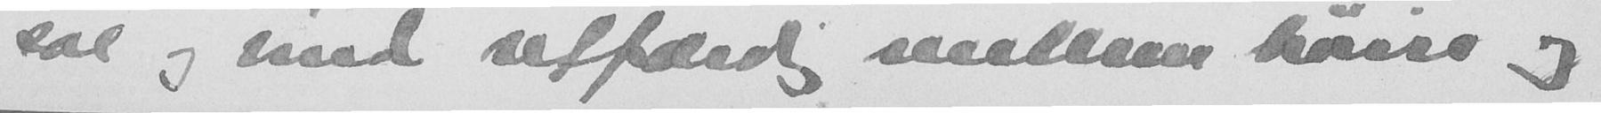sol og vind utferdig mellom bøsse og
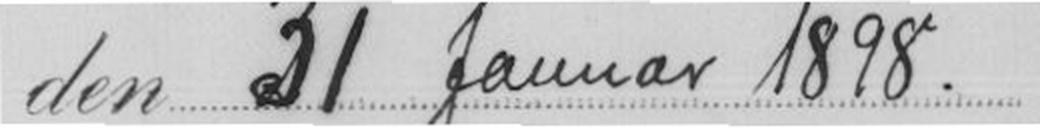den 31 Januar 1898.
